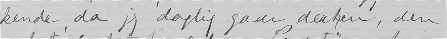kende, da jeg daglig gaar derhen, den
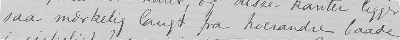saa mærkelig langt fra hverandre baade
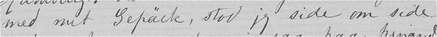med mit Gepäck, stod jeg side om side
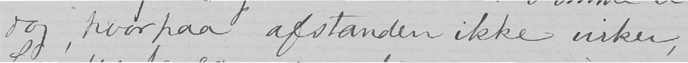dog, hvorpaa afstanden ikke virker,
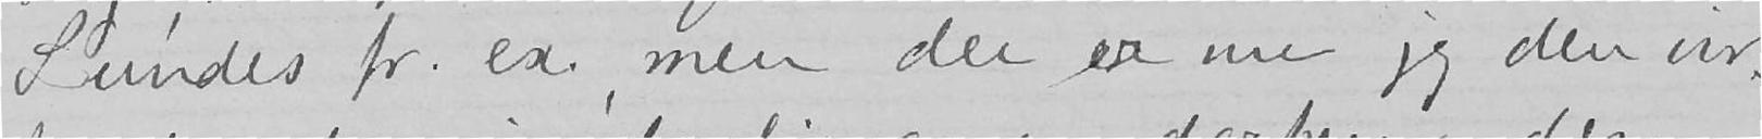Lundes fr. ex., men der er nu jeg den vir-
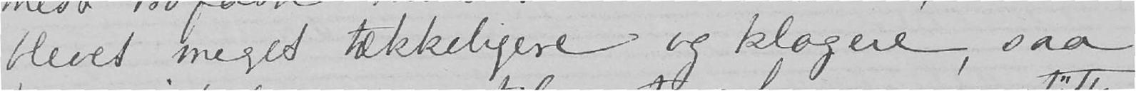blevet meget tækkeligere og klogere, saa
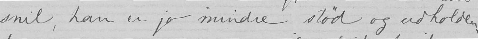snil, han er jo mindre stød og udholden-
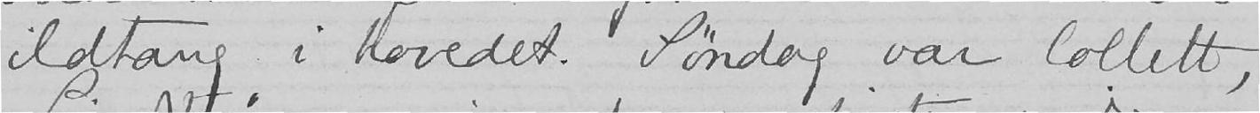ildtang i hovedet. Søndag var Collett,
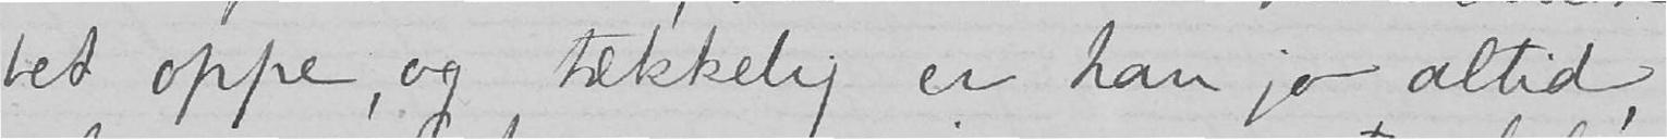bet oppe, og tækkelig er han jo altid,
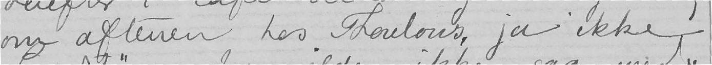om aftenen hos Thoulows, ja ikke
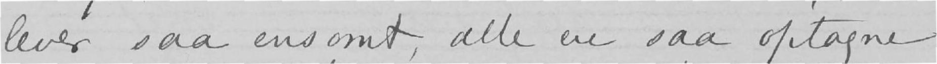lever saa ensomt, alle ere saa optagne
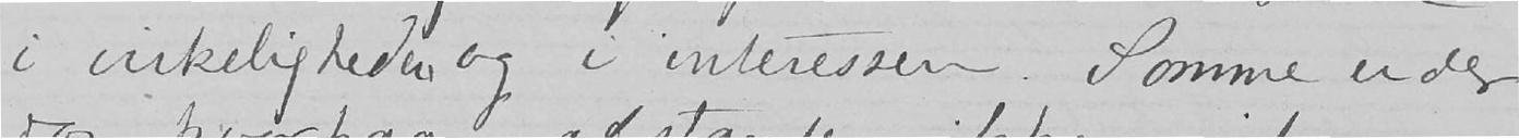i virkeligheden og i interessen. Somme er der
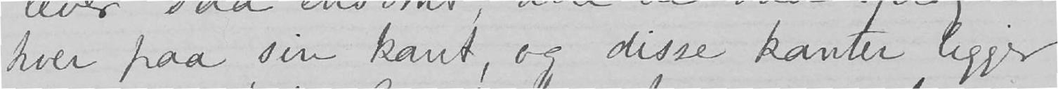hver på sin kant, og disse kanter ligger
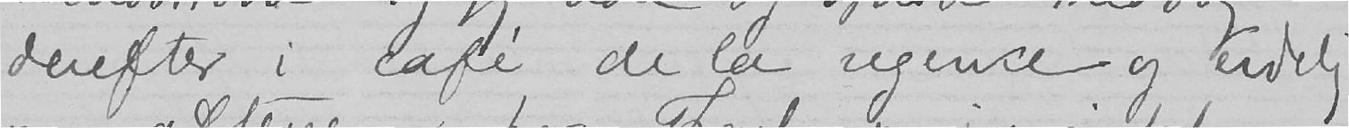derefter i Café de la regence og endelig
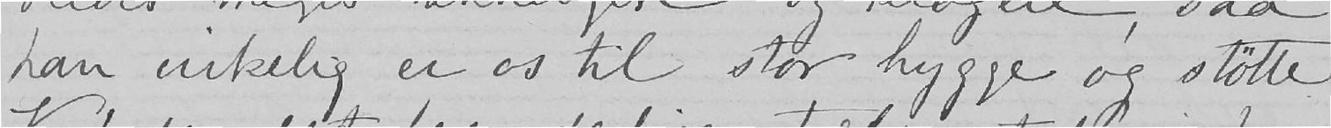han virkelig er os til stor hygge og støtte
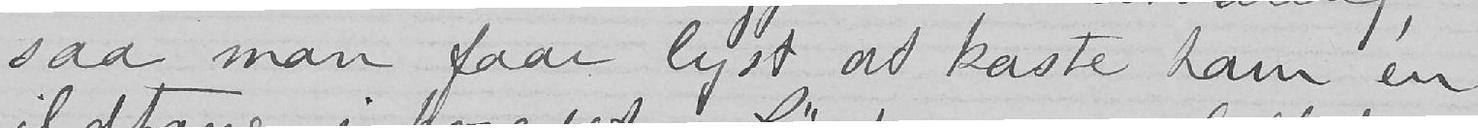saa man faar lyst at kaste ham en
